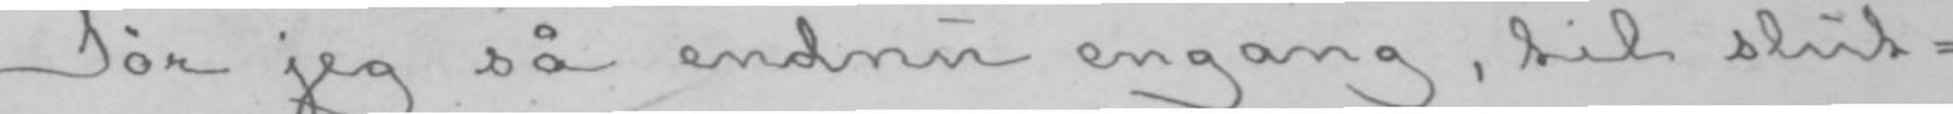Tør jeg så endnu engang, til slut-
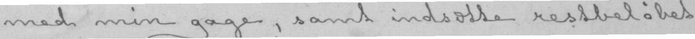med min gage, samt indsætte restbeløbet
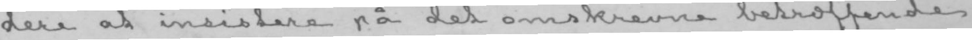dere at insistere på det omskrevne betræffende
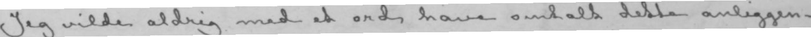Jeg vilde aldrig med et ord have omtalt dette anliggen-
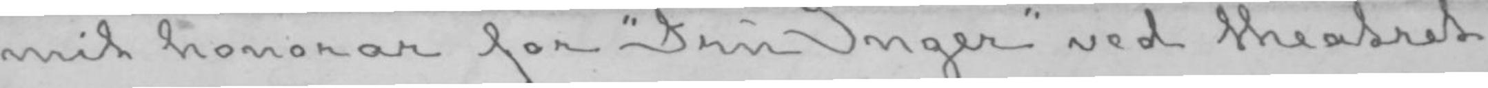mit honorar for «Fru Inger» ved theatret,
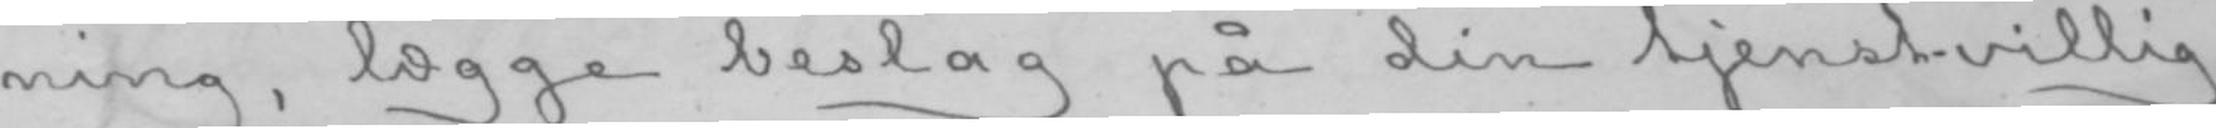ning, lægge beslag på din tjenstvillig-
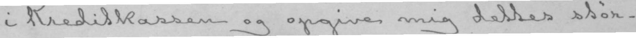i Kreditkassen og opgive mig dettes stør-
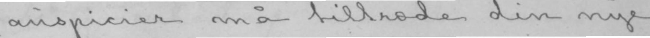ge auspicier må tiltræde din nye
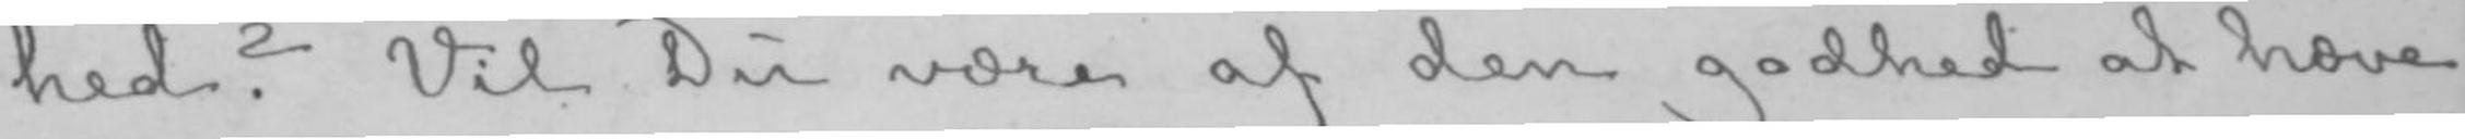hed? Vil Du være af den godhed at hæve
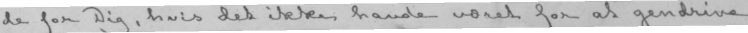de for Dig, hvis det ikke havde været for at gendrive
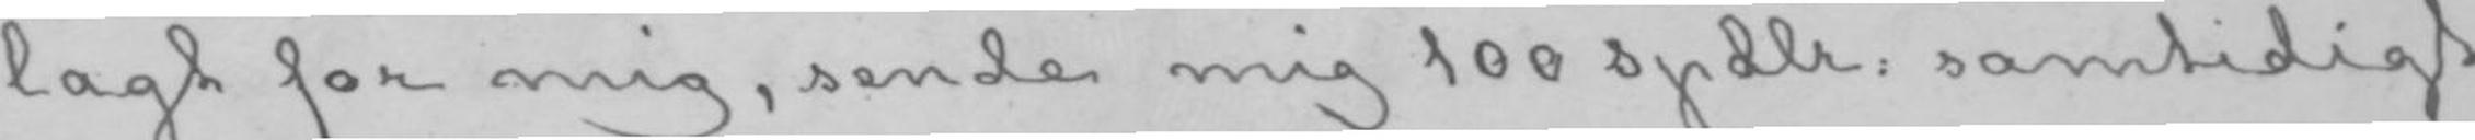lagt for mig, sende mig 100 spdlr: samtidigt
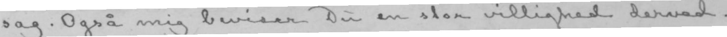sag. Også mig beviser Du en stor villighed derved.
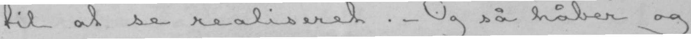til at se realiseret.- Og så håber og
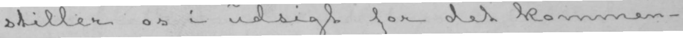stiller os i udsigt for det kommen-
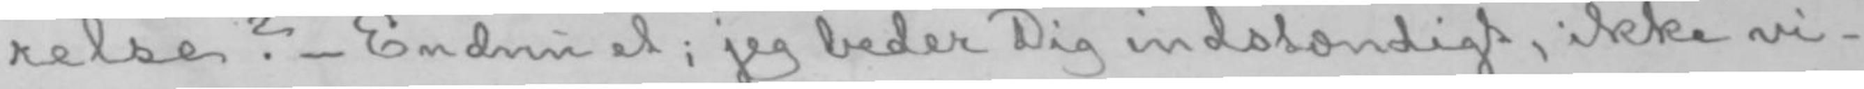relse?- Endnu et; jeg beder Dig indstændigt, ikke vi-
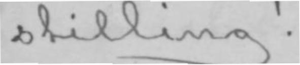stilling!
